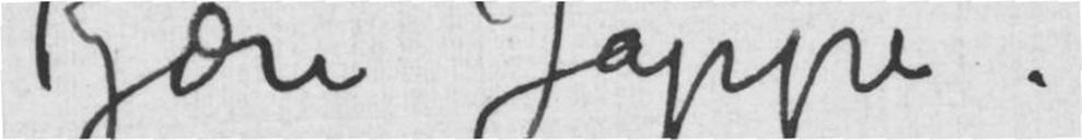Kjære Jappe!
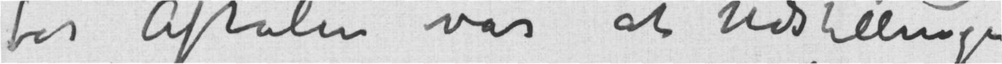for Avtalen var at Udstillingen
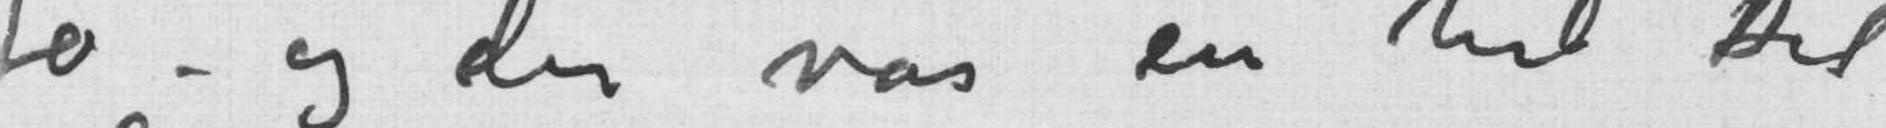få – og der var en hel Del
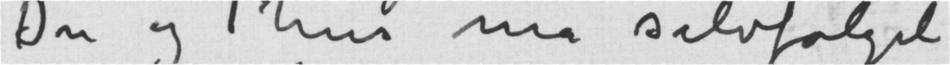Du og Thiis må selvfølgeli
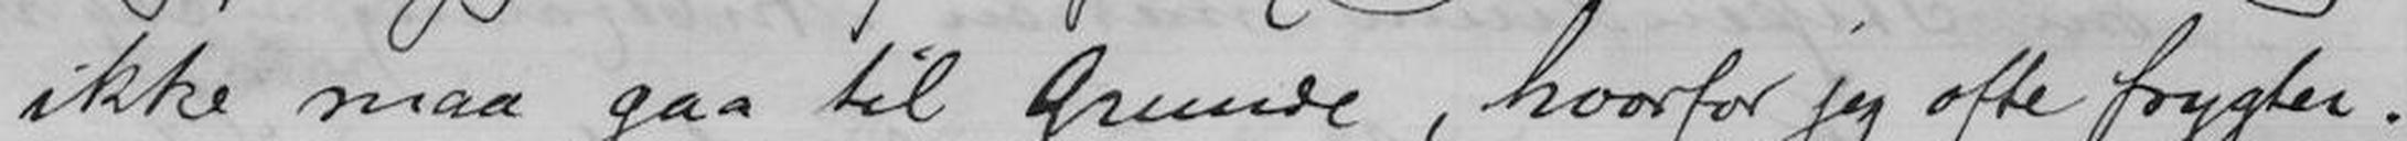ikke maa gaa til Grunde, hvorfor jeg ofte frygter.
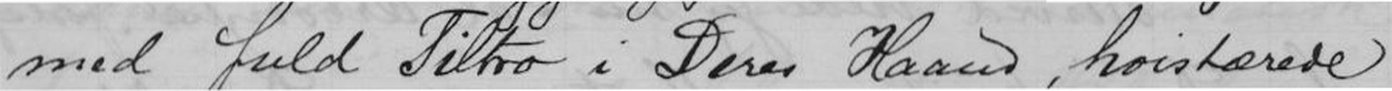med fuld Tiltro i Deres Haand, høistærede
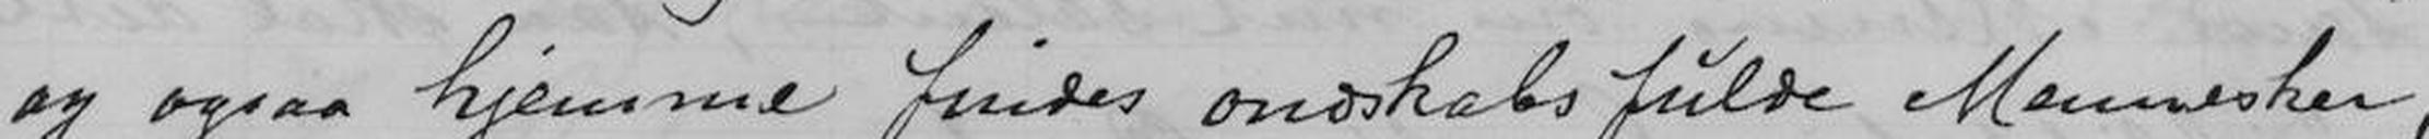og ogsaa hjemme findes ondskabsfulde Mennesker,
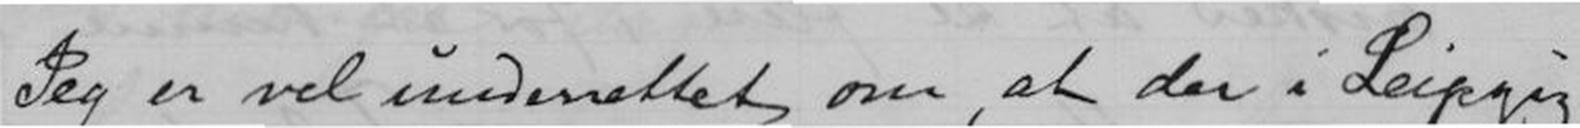Jeg er vel underrettet om, at der i Leipzig
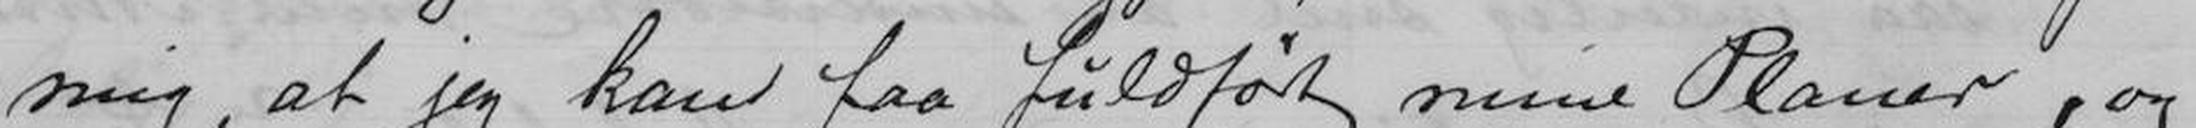mig, at jeg kan faa fuldført mine Planer, og
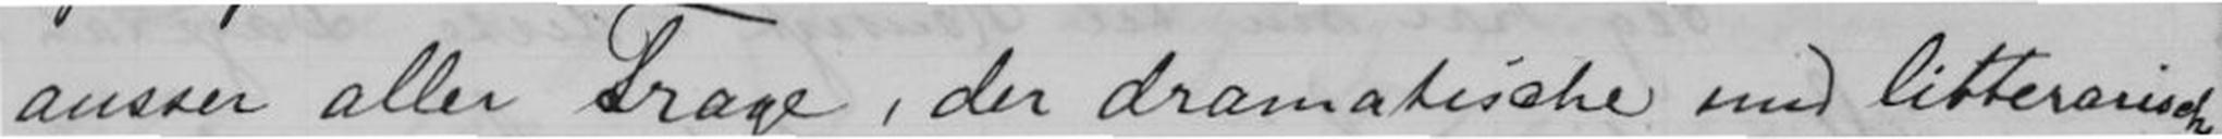ausser aller Frage, der dramatische und litterarische
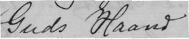Guds Haand. -
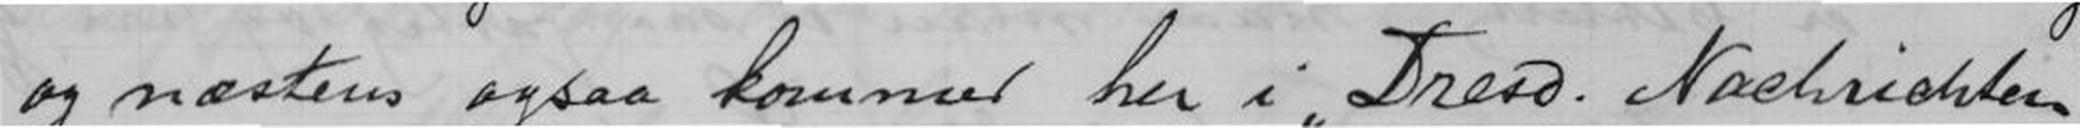og nestens ogsaa kommer her i "Dresd. Nachrichten".
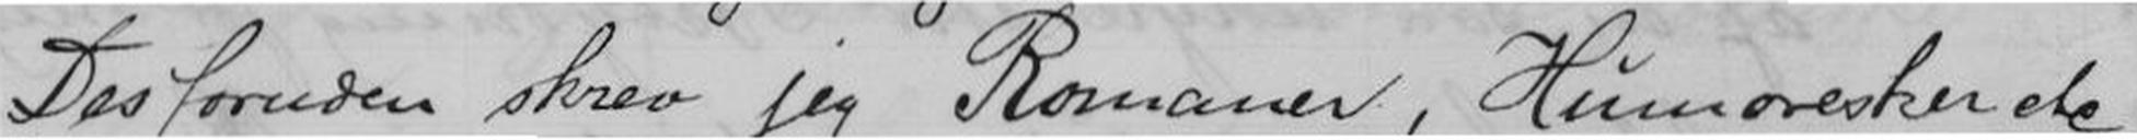Desforuden skrev jeg Romaner, Humoresker etc,
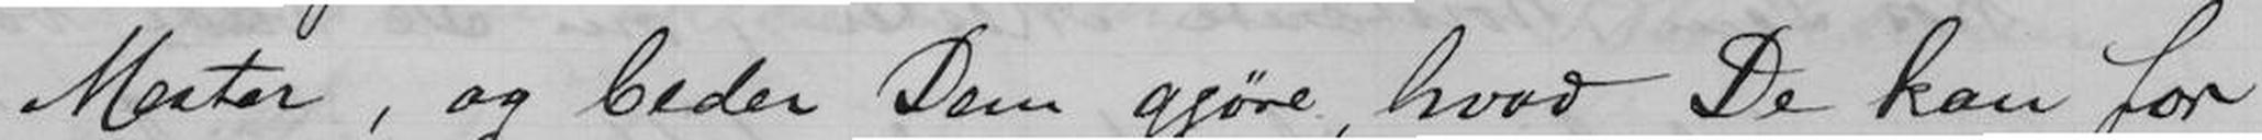Mester, og beder Dem gjøre, hvad De kan for
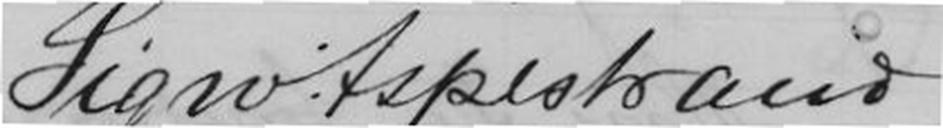Sigw. Aspestrand
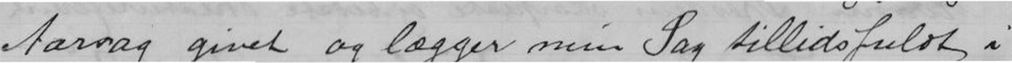Aarsag givet og lægger min Sag tillidsfuldt i
